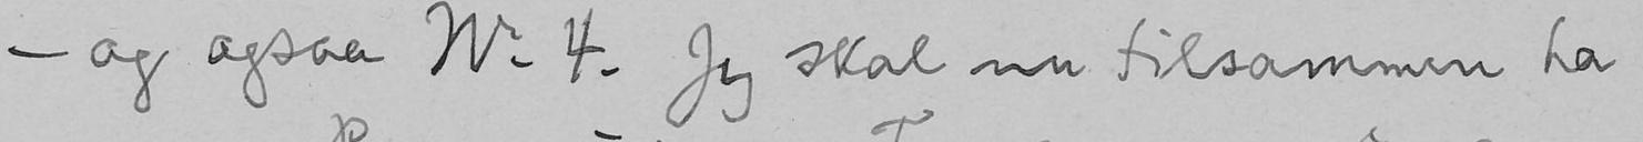- og ogsaa Nr 4. Jeg skal nu tilsammen ha
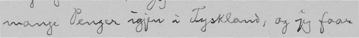mange Penger igjen i Tyskland, og jeg faar
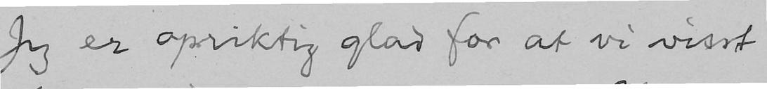Jeg er opriktig glad for at vi visst
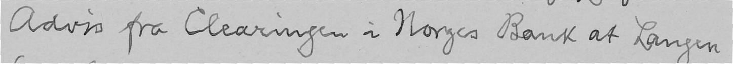Advis fra Clearingen i Norges Bank at Langen
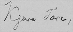Kjære Tore,
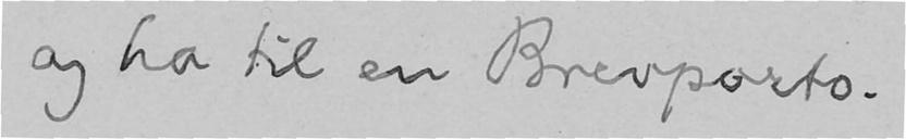og ha til en Brevporto.
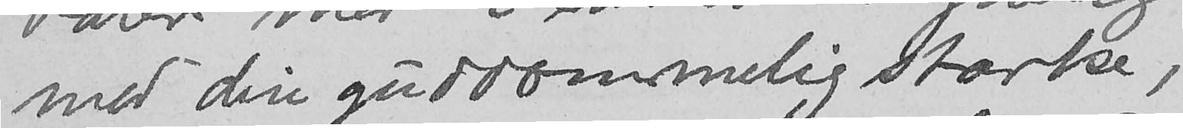med din guddommelig stærke,
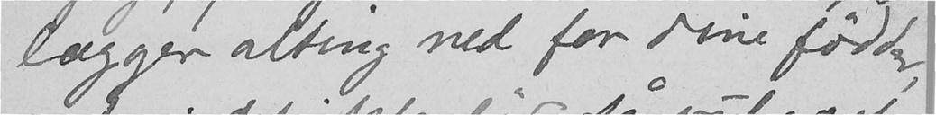lægger alting ned for dine fødder,
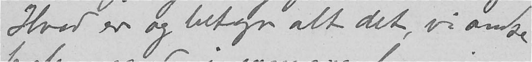Hvad er og betyr alt det, vi andre
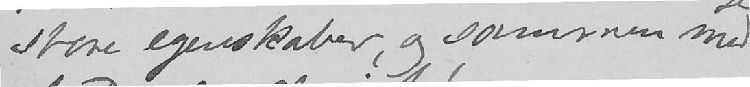store egenskaber, og sammen med
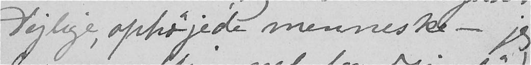Dejlige, ophøjede menneske - jeg
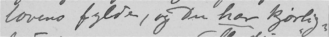lovens fylde, og Du har kjærlig-
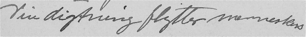Din digtning flytter menneskers
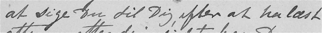at sige Du til Dig, efter at ha læst
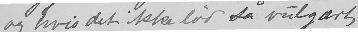og hvis det ikke lød så vulgært,
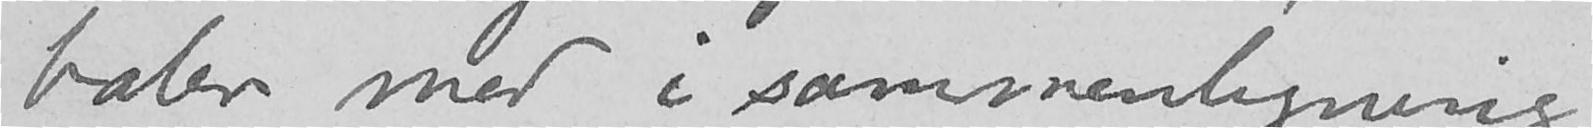baler med i sammenligning
